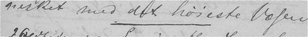nesket med det høieste Væsen
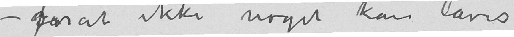– forat ikke noget kan laves
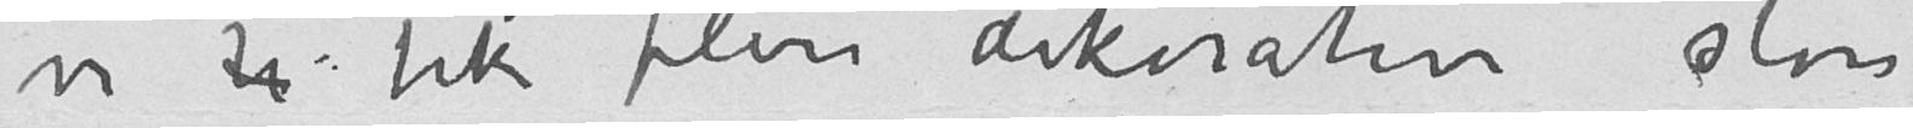vi h fik flere dekorative store
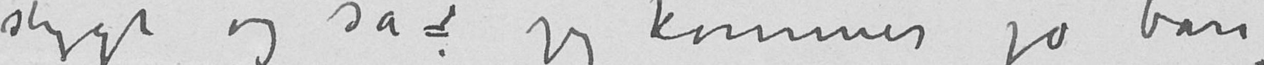stygt og sa: jeg kommer jo bare
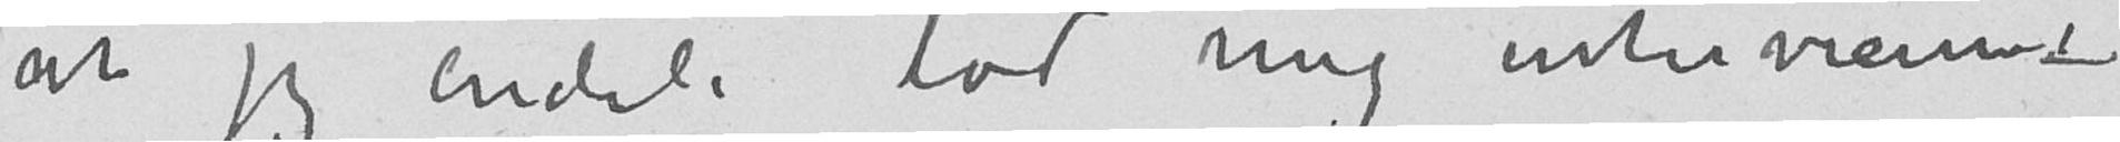at jeg endeli lod mig interviewe –
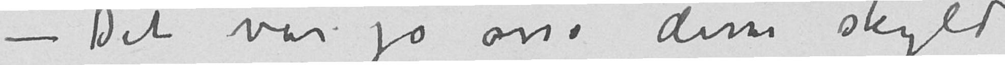– Det var jo osså disses skyld
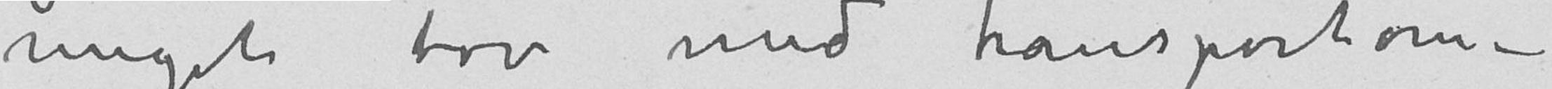noget tøv med transportom-
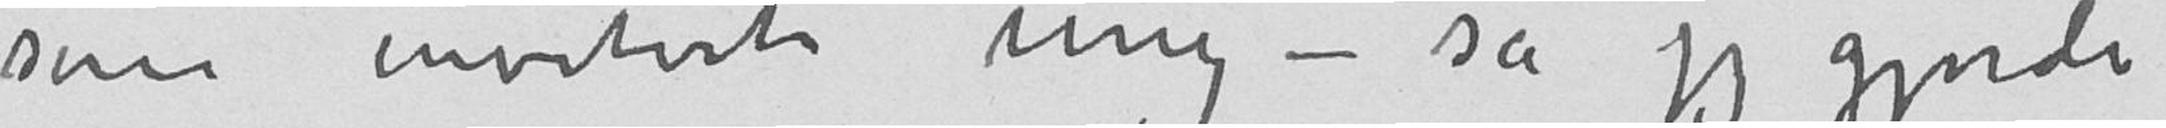som inviterte mig – så jeg gjorde
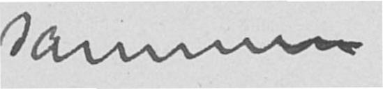sammen
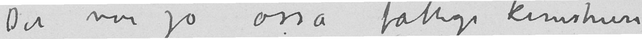Det var jo osså fattige kunstnere
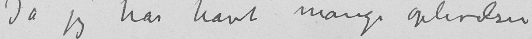Ja jeg har havt mange oplevelser
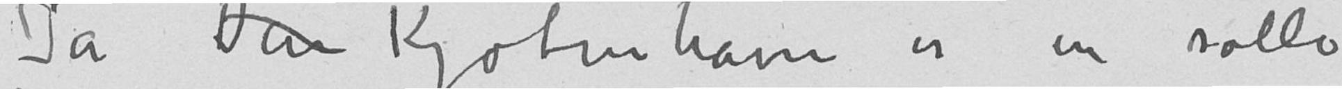Ja Den Kjøbenhavn er en solle
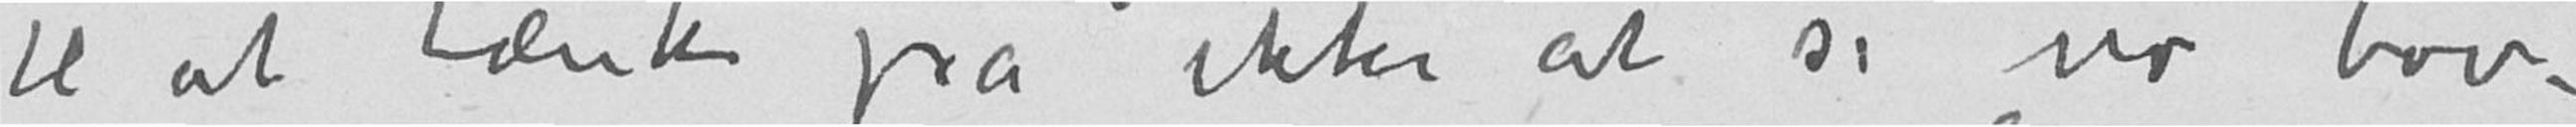til at tænke på ikke at si no tøv –
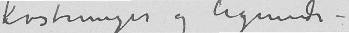kostninger og lignende –
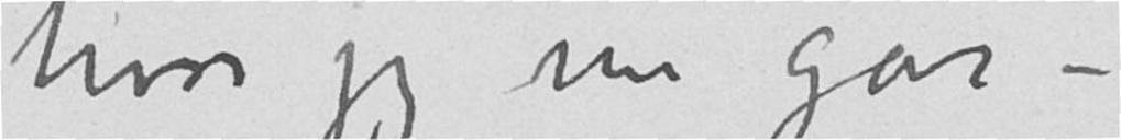hvor jeg nu går –
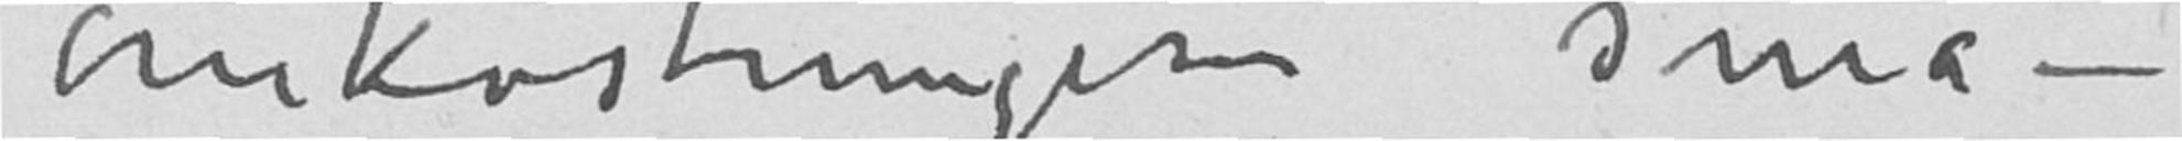omkostningerne små –
